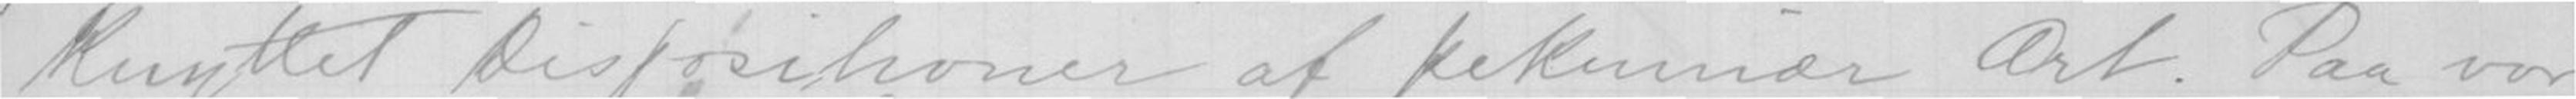knyttet Dispositioner af pekuniær Art. Paa vor
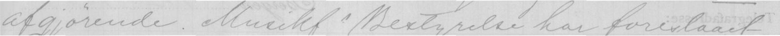afgjørende. Musikfs Bestyrelse har foreslaaet
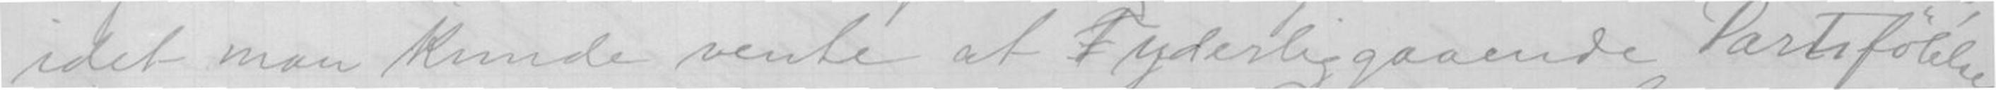idet man kunde vente at T yderliggaaende Partifølelse
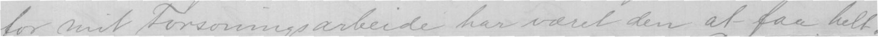for mit Forsoningsarbeide har været den af faa helt og
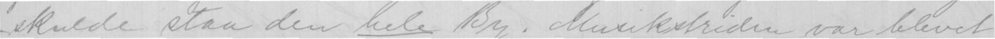skulde staa den hele By. Musikstriden var blevet
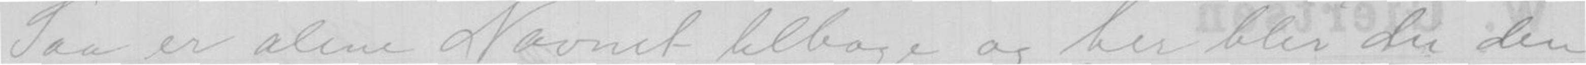Saa er alene Navnet tilbage og her blir du den
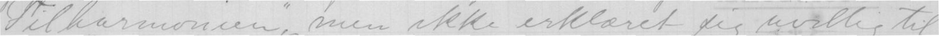Filharmonien men ikke erklæret sig uvillig til
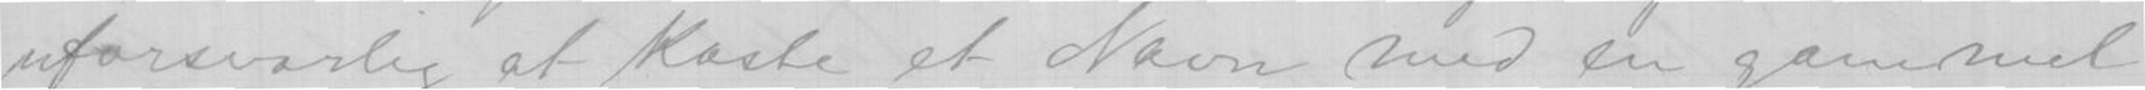uforsvarlig at kaste et Navn med en gammel
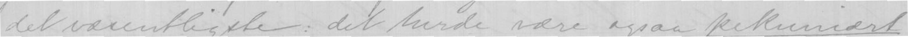det væsentligste: det turde være ogsaa pekunuært
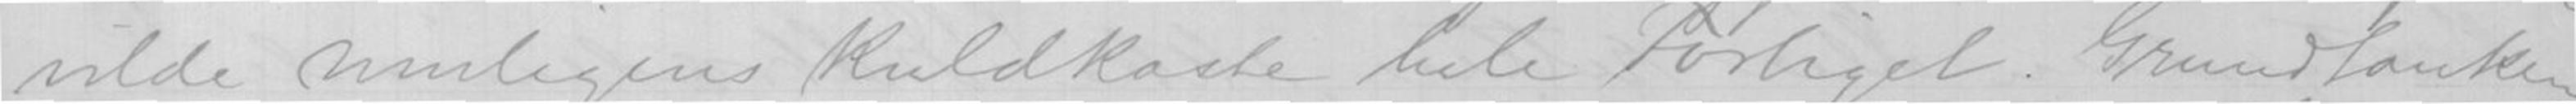vilde muligens Kuldkaste hele Forliget. Grundtanken
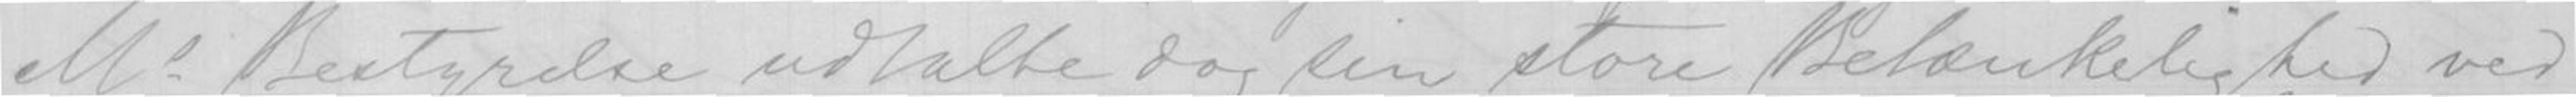Ms Bestyrelse udtalte dog sin store Betænkelighed ved
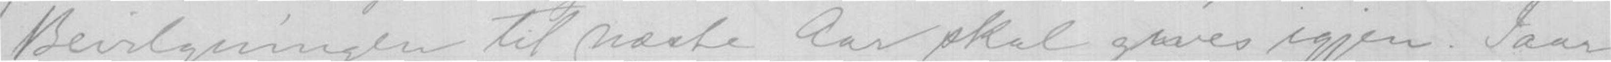Bevilgningen til næste Aar skal gives igjen. Iaar
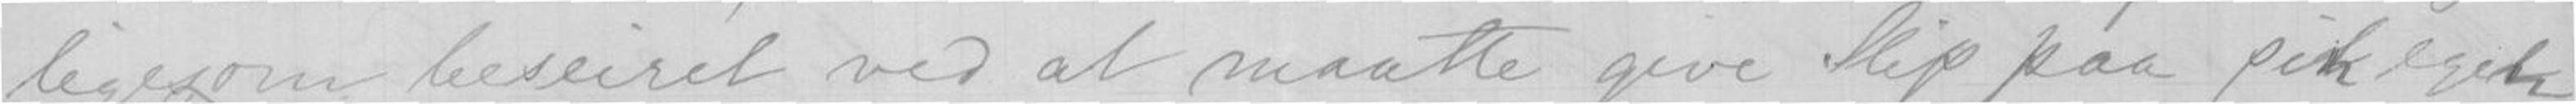ligesom beseiret ved at maatte give Slip paa sin egen
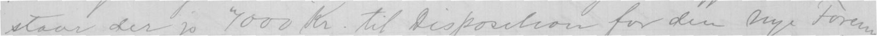staar der jo 7000 Kr. til Disposition for den nye Forening
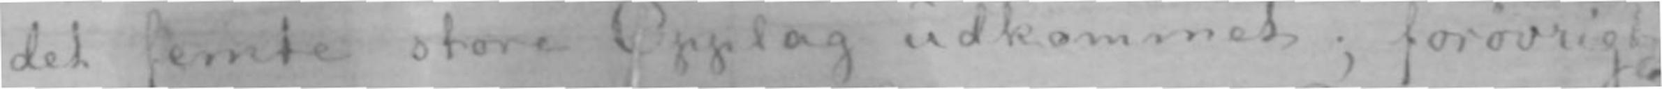det femte store Opplag udkommet; forøvrigt
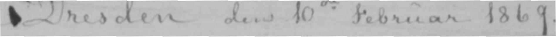Dresden den 10de Februar 1869.-
